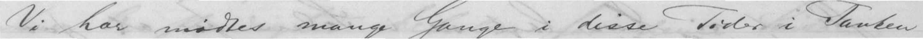Vi har mødtes mange Gange i disse Tider i Tanken
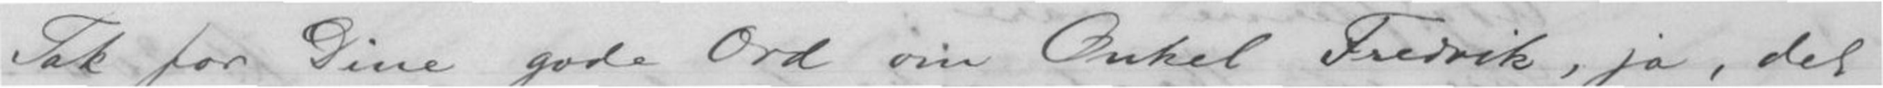Tak for Dine gode Ord om Onkel Fredrik, ja, det
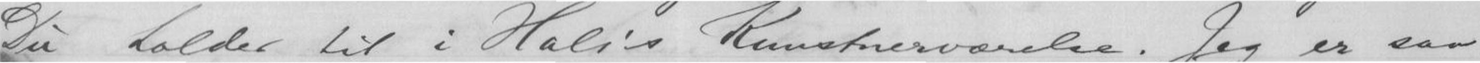Du holder til i Hals's Kunstnerværelse. Jeg er saa
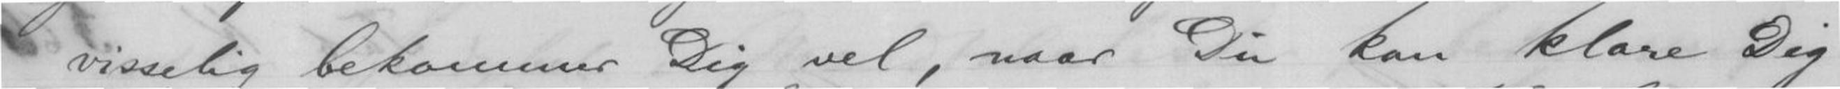visselig bekommer Dig vel, naar Du kan klare Dig
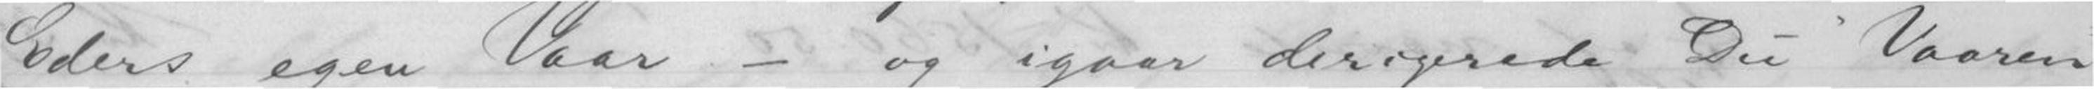Eders egen Vaar - og igaar dirigerede Du 'Vaaren'
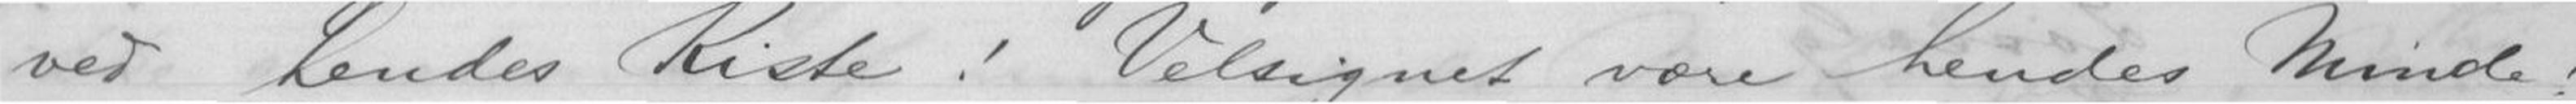ved hendes Kiste! Velsignet være hendes Minde!
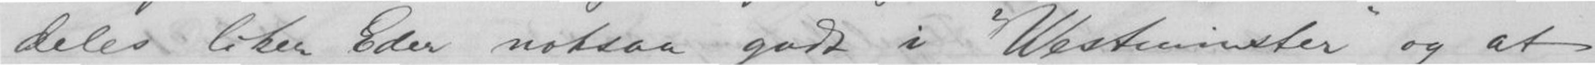deles liker Eder noksaa godt i "Westmister" og at
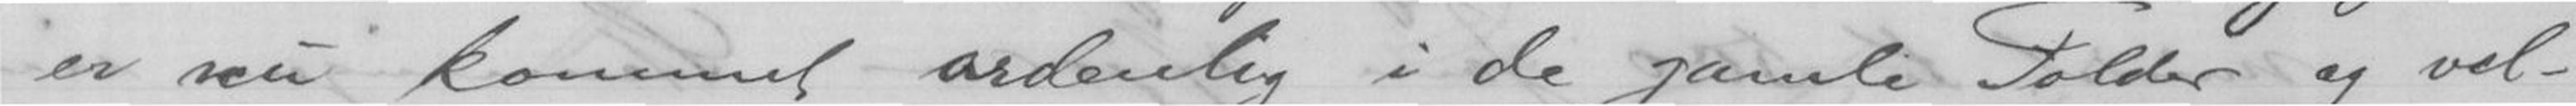er nu kommet ordentlig i de gamle Folder og vel-
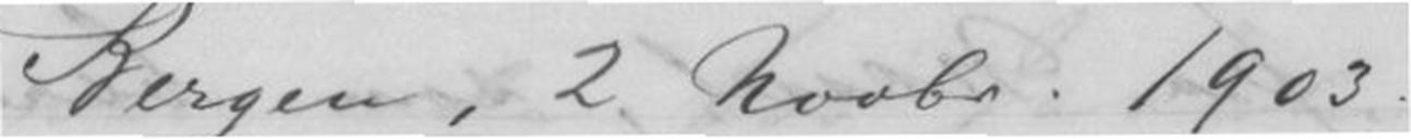Bergen, 2 Novbr. 1903.
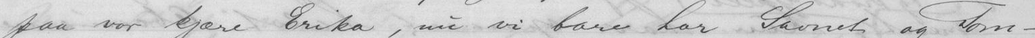paa vor kjære Erika, nu vi bare har Savnet og Tom-
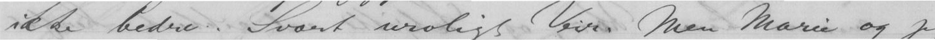ikke bedre. Svært uroligt Veir. Men Marie og jeg
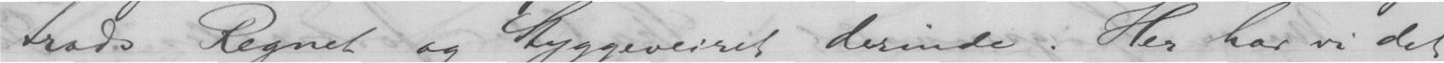trods Regnet og Styggeveiret derinde. Her har vi det
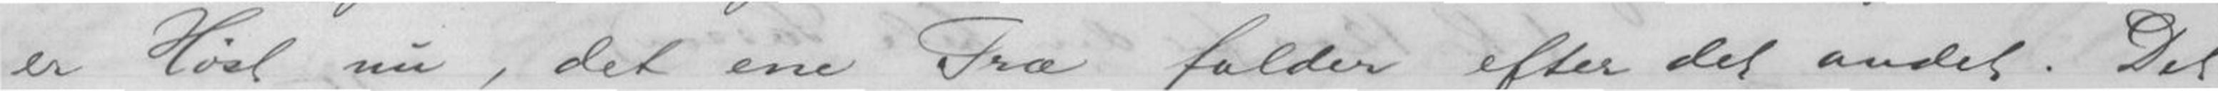er Høst nu, det ene Træ falder efter det andet. Det
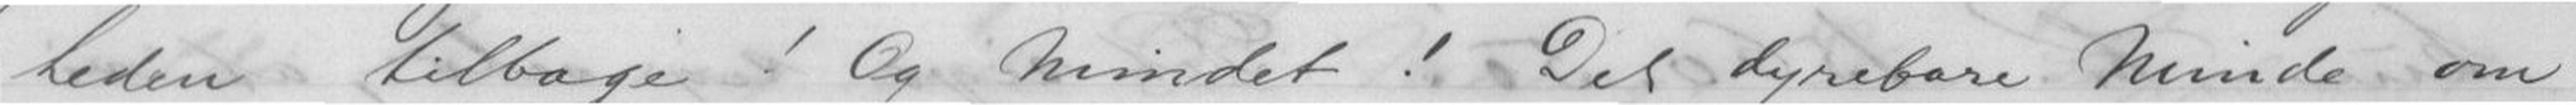heden tilbage! Og Mindet! Det byrebare Minde om
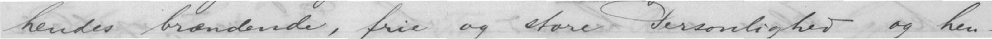hendes brændende, frie og store Personlighed og hen-
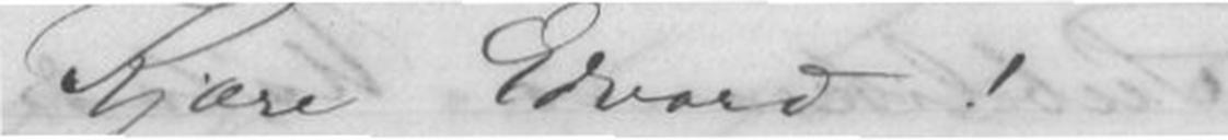Kjære Edvard!
